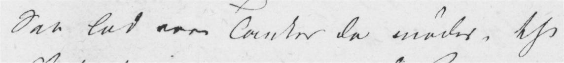Saa lad vore Tanker da mødes, thi
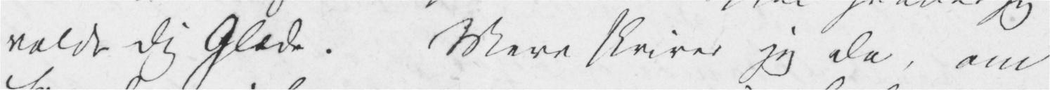volde Dig Glæde. Mere skriver jeg da, om
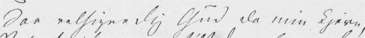Saa velsigne Dig Gud da min kjere,
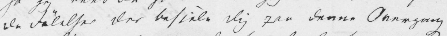de Følelser der besjele Dig paa denne Overgang
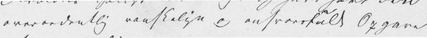overordentlig vanskelige og ansvarsfulde Opgave
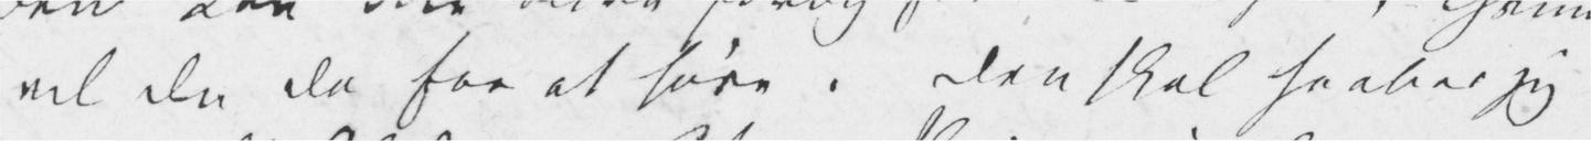vil Du da faa at høre. Den skal haaber jeg
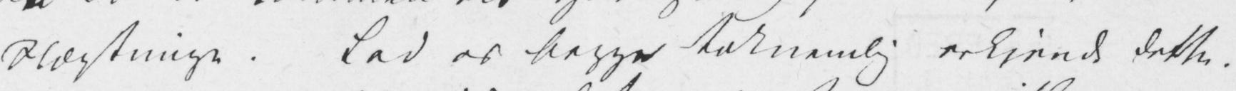Slægtninge. Lad os begge taknemlig erkjende dette.
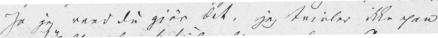Ja jeg veed du gjør det, jeg tvivler ikke paa
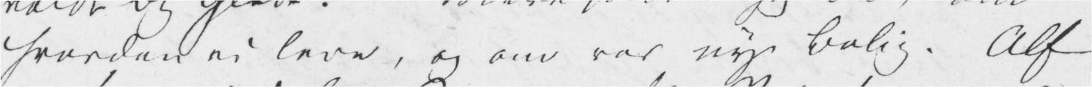hvordan vi leve, og om vor nye Bolig. Alf
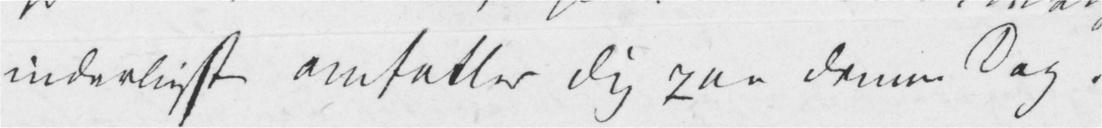inderligst omfatter Dig paa denne Dag.
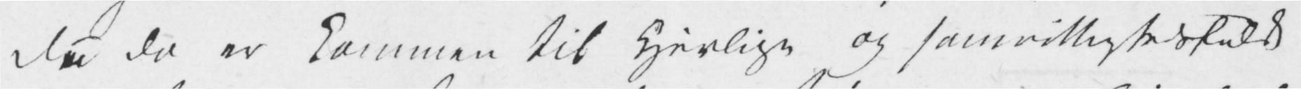Du da er kommen til kjerlige og samvittighedsfulde
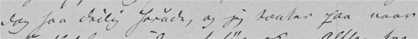dog saa deilig herude, og jeg tænker paa naar
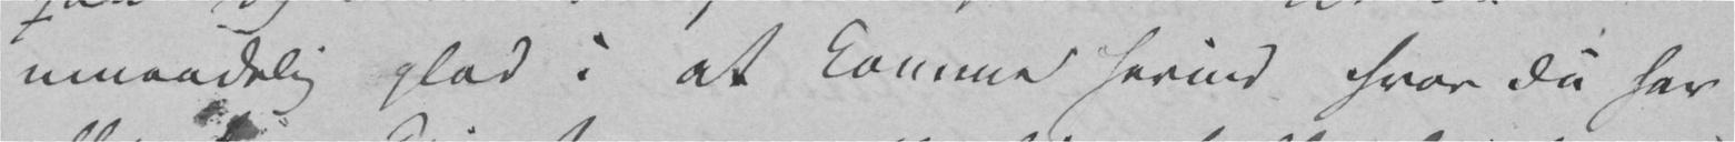umaadelig glad i at komme herind hvor Du har
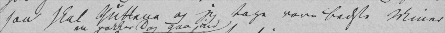saa skal Guttene og jeg tage vore bedste Miner
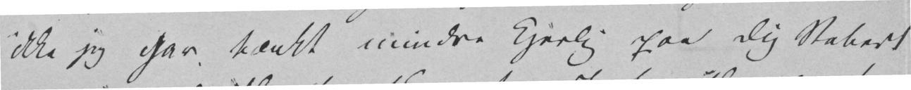ikke jeg har tænkt mindre kjerlig paa Dig Robert
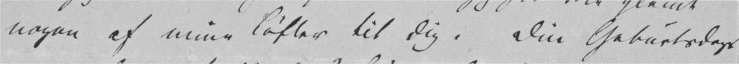nogen af mine Løfter til Dig. Din Geburtsdagsgave
